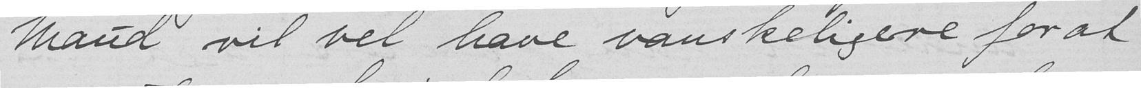Maud vil vel have vanskeligere for at
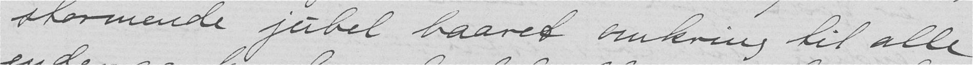stormende jubel baaret omkring til alle
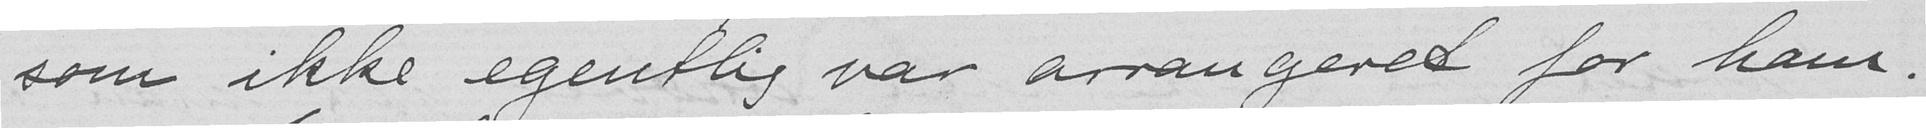som ikke egentlig var arrangeret for ham.
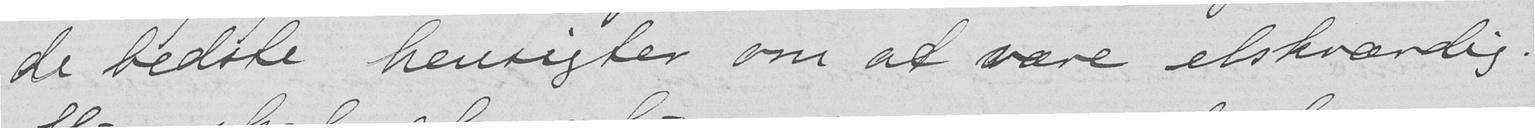de bedste hensigter om at være elskværdig.
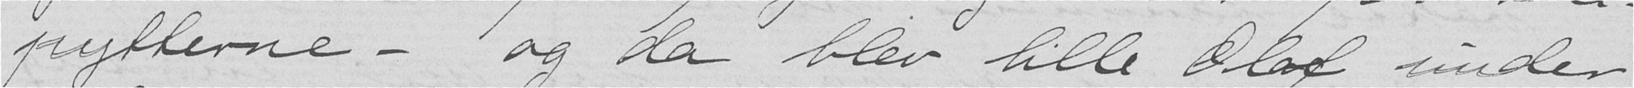pytterne – og da blev lille Olaf under
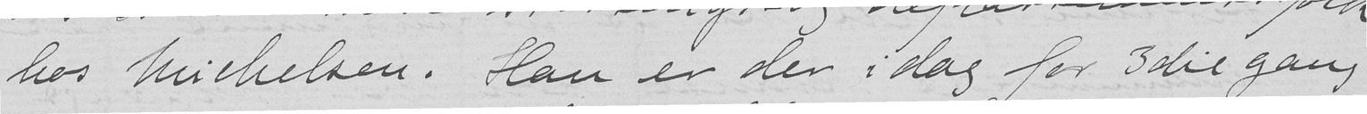hos Michelsen. Han er der i dag for 3die gang
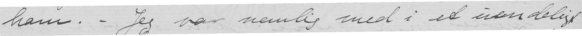ham. – Jeg var nemlig med i et uendeligt
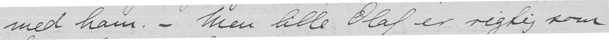med ham. – Men lille Olaf er rigtig som
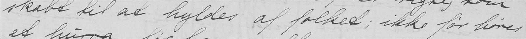skabt til at hyldes af folket; ikke før høres
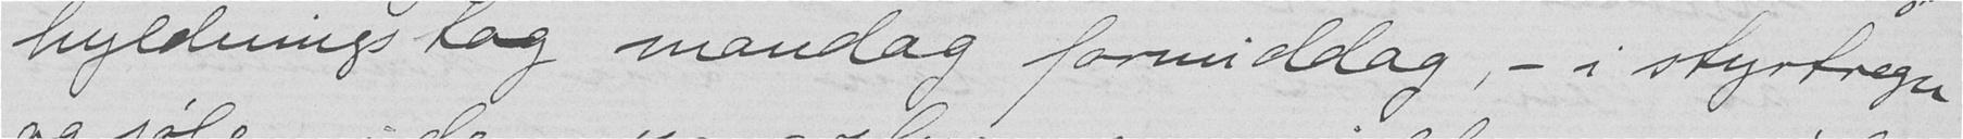hyldningstog mandag formiddag, – i styrtregn
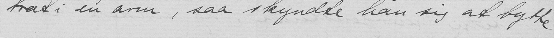træt i en arm, saa skyndte han sig at bytte
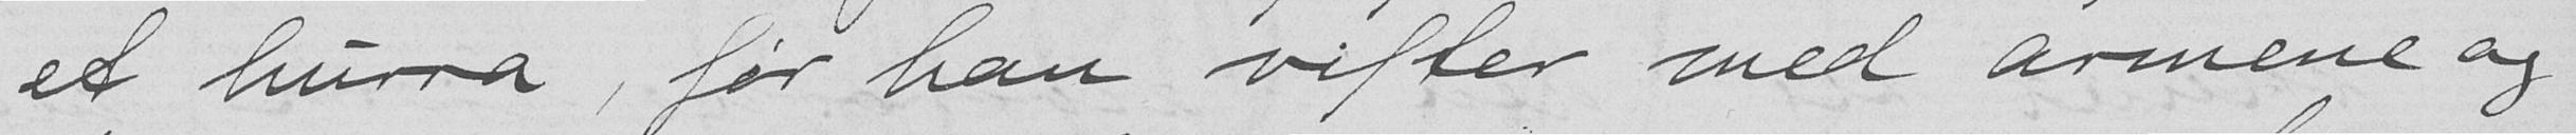et hurra, før han vifter med armene og
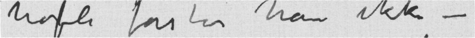høfli forstår han ikke –
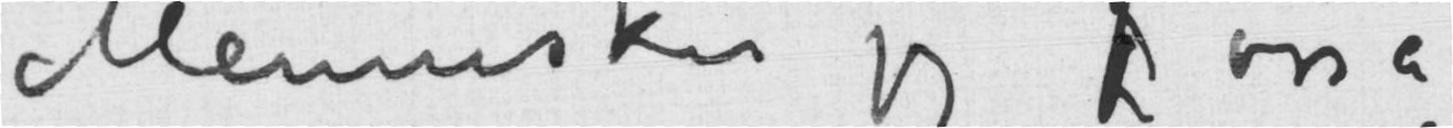Mennesker jegosså
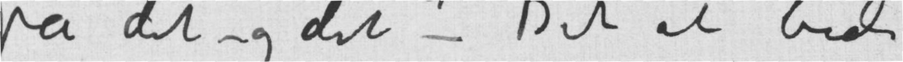på det – og det» – Det at bede
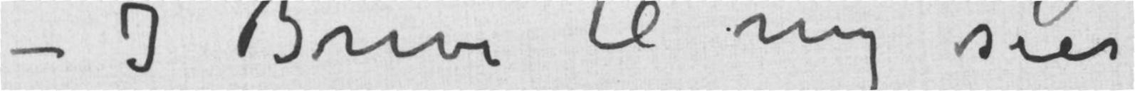– I Breve til mig sier
